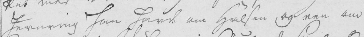Jernring han havde om Halsen og een om
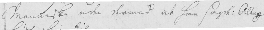Menneske uden dermed at han sagde: Alting
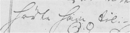hørte ham til. -
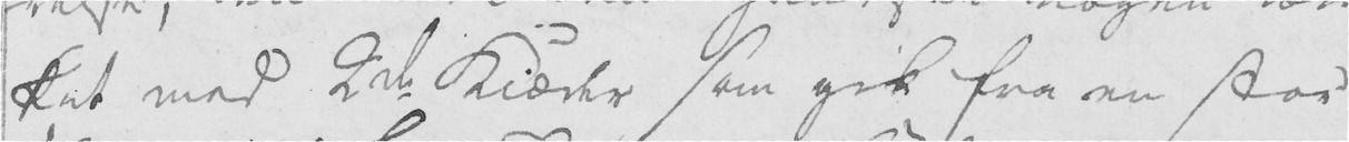ket med 2de Kiæder som gik fra en stor
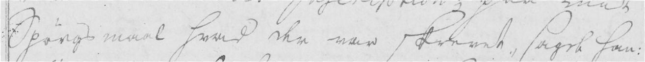Spørgsmaal hvad der var skrevet, sagde han:
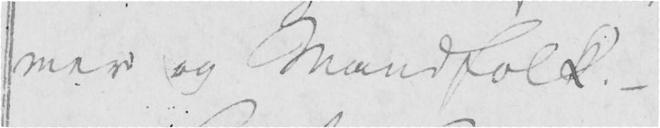mer og Mandfolk. -
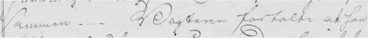sammen. - Vogteren fortalte at han
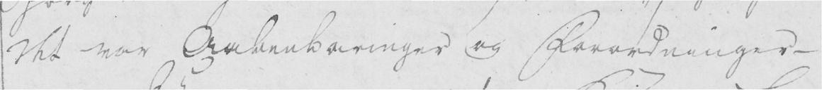det var Aabenbaringer og Forordninger -
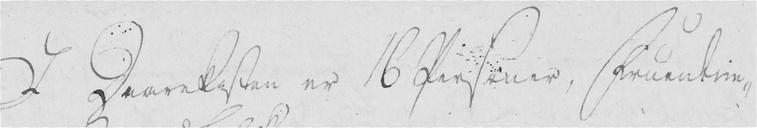I Daarekisten er 16 Personer, Fruentim-
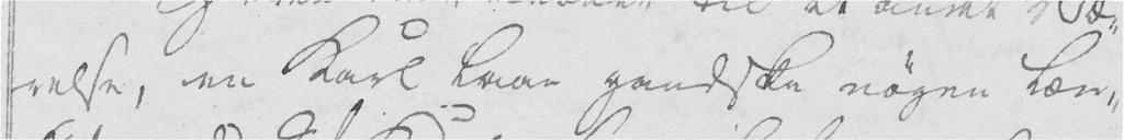relse, en Karl laae gandske nøgen læn-
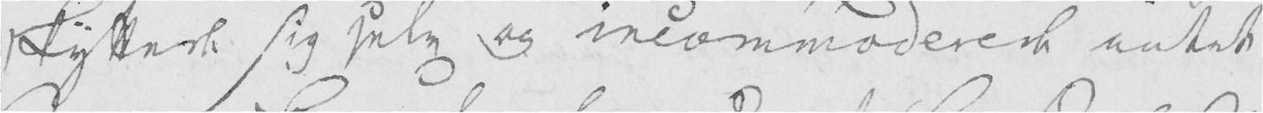skyttede sig selv og incommoderede intet
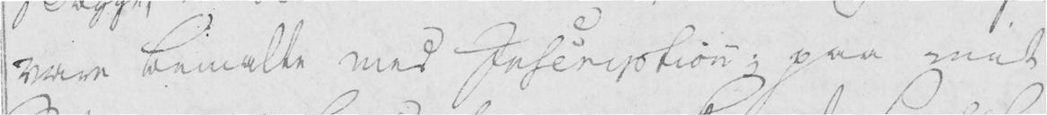vare bemalte med Inscription; paa mit
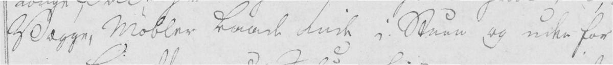Vægge, Møbler baade inde i Stuen og uden for
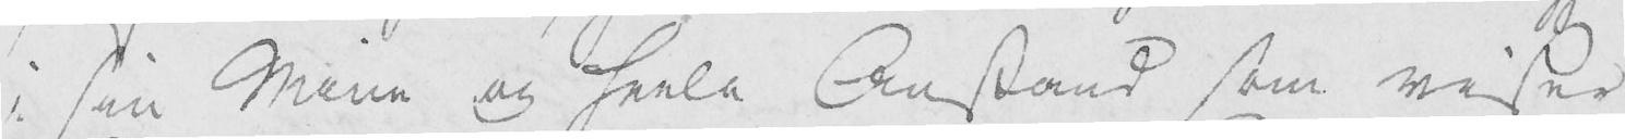i sin Mine og heele Anstand som viser
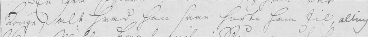Konge, alt hvad han saae hørte ham til, alting
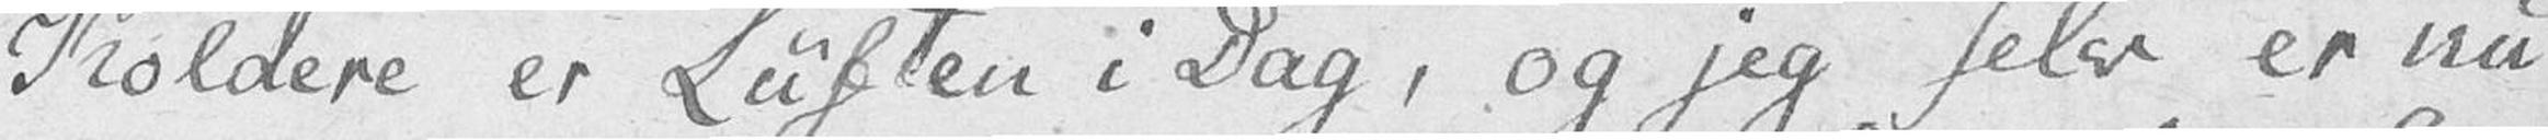Koldere er Luften i Dag, og jeg selv er nu
